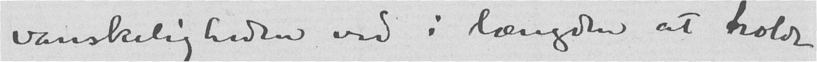vanskeligheden ved i længden at holde
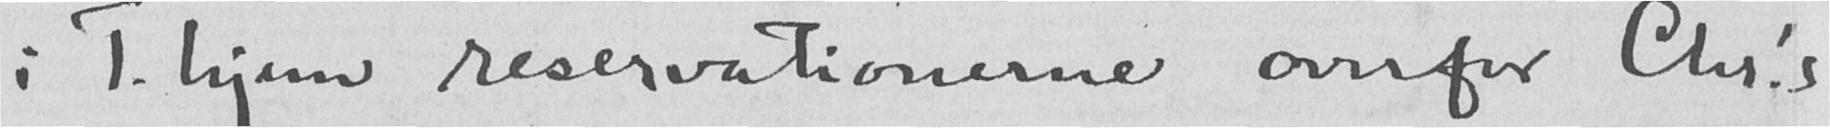i T. hjem reservationerne overfor Chr.´s
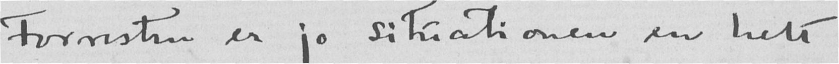Forresten er jo situationen en helt
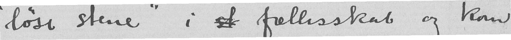"løse stene" i sl fællesskab og kom
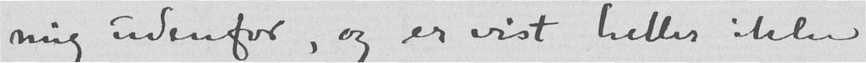mig udenfor, og er vist heller ikke
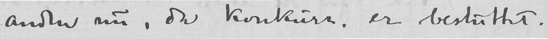anden nu, da konkurr. er besluttet.
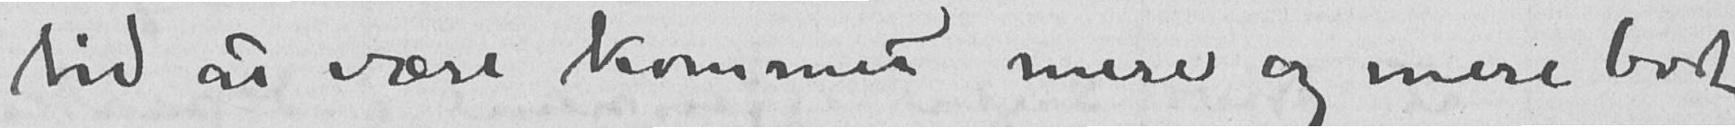tid at være kommet mere og mere bort
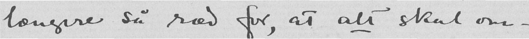længere så ræd for, at alt skal om-
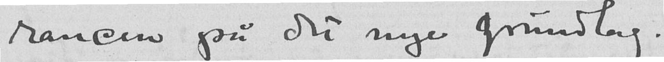rancen på det nye grundlag.
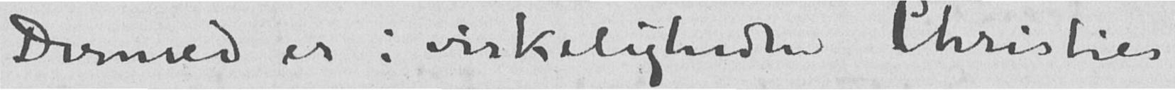Dermed er i virkeligheden Christies
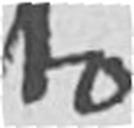to
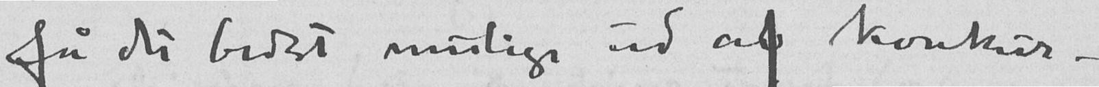få det bedst mulige ud af konkur-
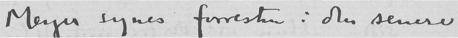Meyer synes forresten i den senere
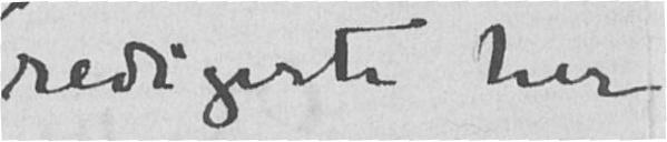redigerte her
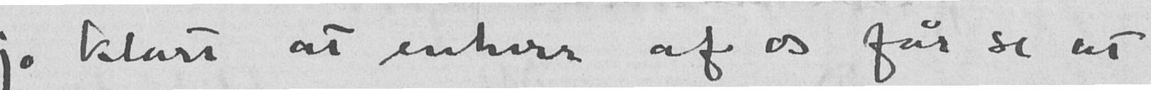jo klart at enhver af os får se at
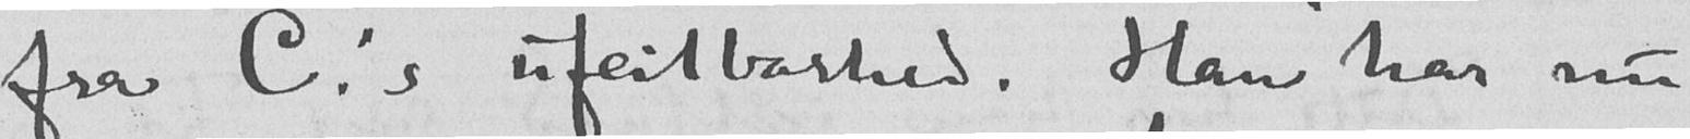fra C.´s ufeilbarhed. Han har nu
